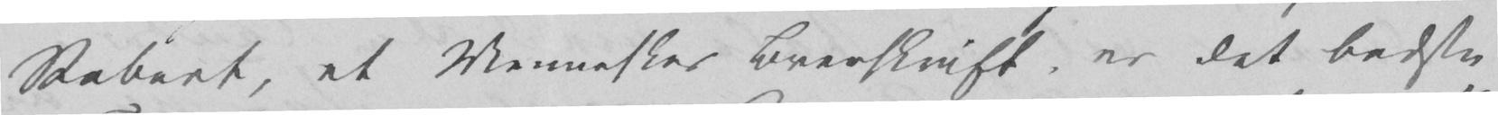Robert, et Menneskes Brevskrift, er det bedste
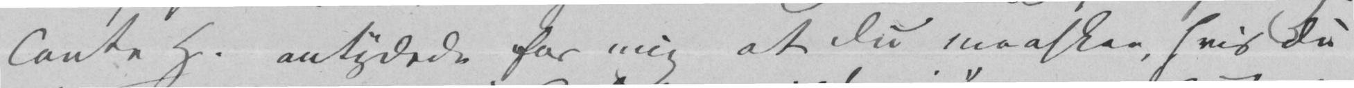Tante H. antydede for mig at Du maaskee, hvis Du
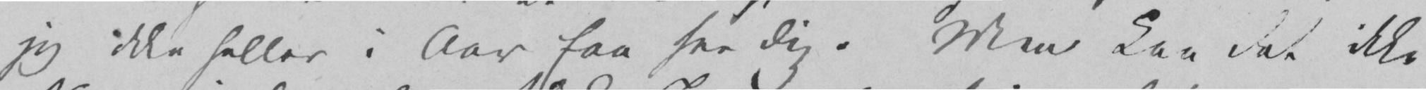jeg ikke heller i Aar faa see Dig. Men kan det ikke
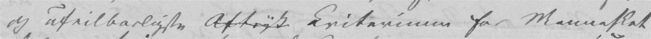og ufeilbarligste Aftryk Kriterium for Mennesket
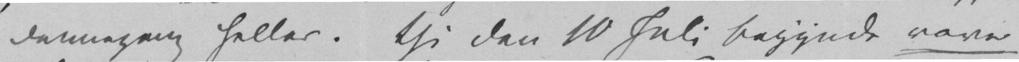dennegang heller. thi den 10 Juli begynde vore
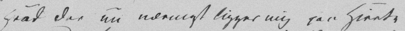Hvad der nu nærmest ligger mig paa Hjerte
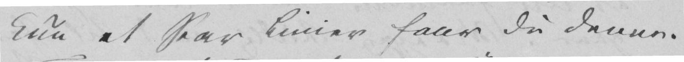Kun et Par Linier faar Du denne
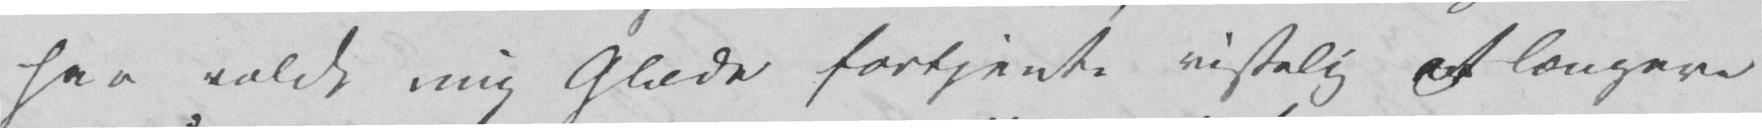har voldt mig Glæde fortjente visselig et længere
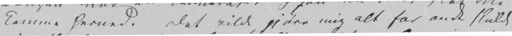komme herned. Det vilde gjøre mig alt for ondt skulde
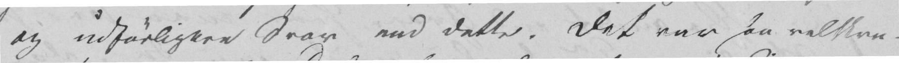og udførligere Svar end dette. Det var saa velskre-
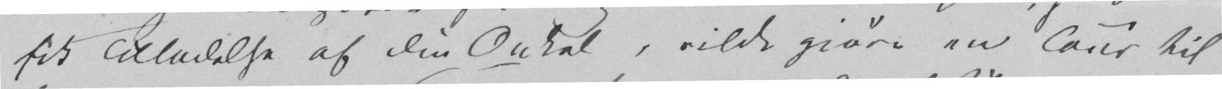fik Tilladelse af Din Onkel, vilde gjøre en Tour til
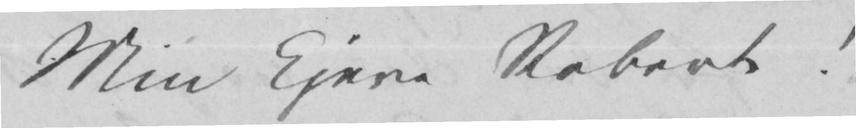Min kjere Robert!
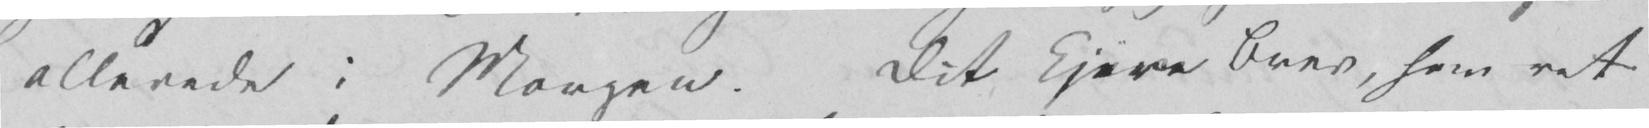allerede i Morgen. Dit kjere Brev, som ret
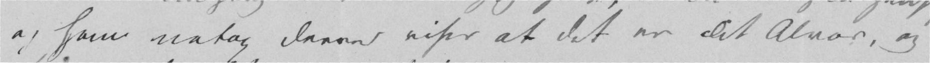og som netop derved viser at det er Dit Alvor, og
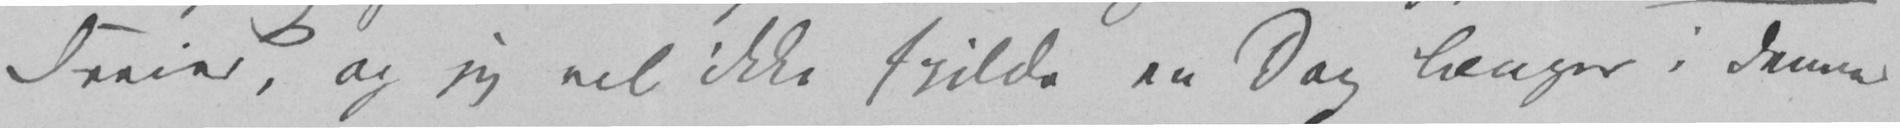Ferier, og jeg vil ikke spilde en Dag længer i denne
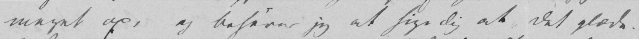meget op, og behøver jeg at sige Dig at det glæde-
